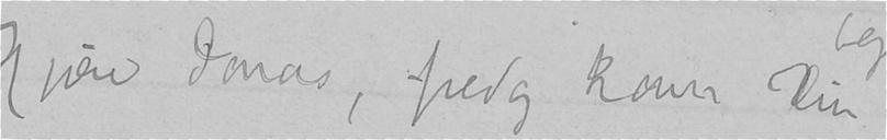Kjære Jonas, fredag kom Din
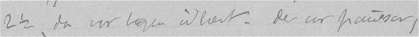2½, da var begge udlæst. Der var pauser
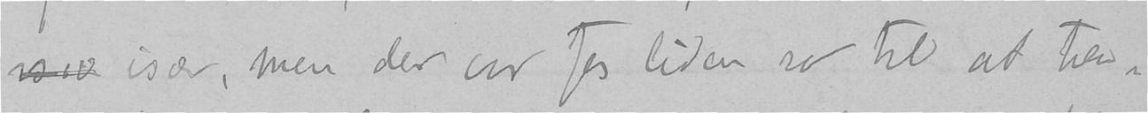sin især, men der var for liden ro til at ten-
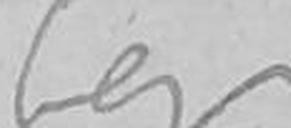bog
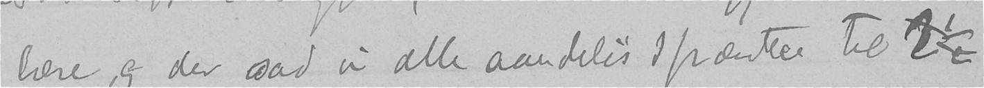læse, og der sad vi alle aandeløs spændte til 2½
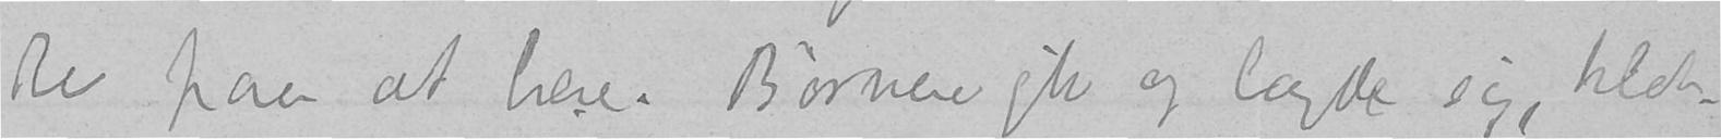ke paa at læse. Børnene gik og lagde sig, klok-
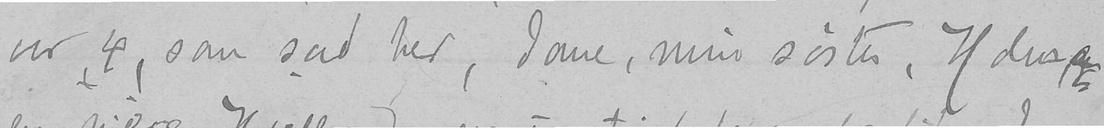var 4, som sad her, Jane, min søster, Holm,
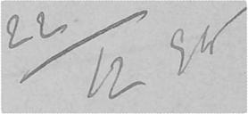22/12 95
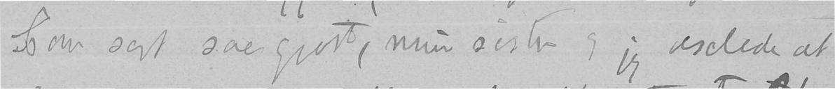Som sagt saa gjort, min søster og jeg ønskede at
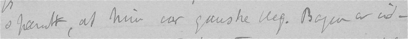spændt, at hun var ganske bleg. Bogen er vid-
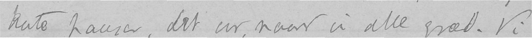korte pauser, det var, naar vi alle græd. Vi
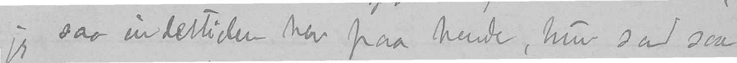jeg saa undertiden her paa hende, hun sad saa
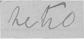hertil
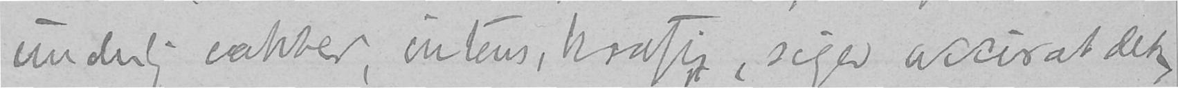underlig vakker, intens, kraftig, siger accurat det
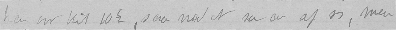ken var blit 10½, saa med et sa en af os, men
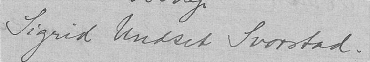Sigrid Undset Svarstad.
